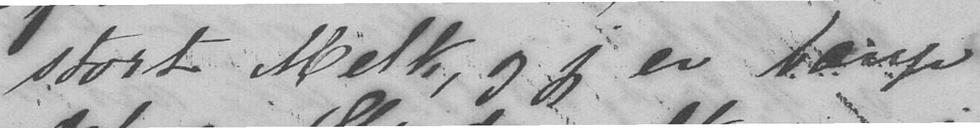stort Melk, og jeg er bange
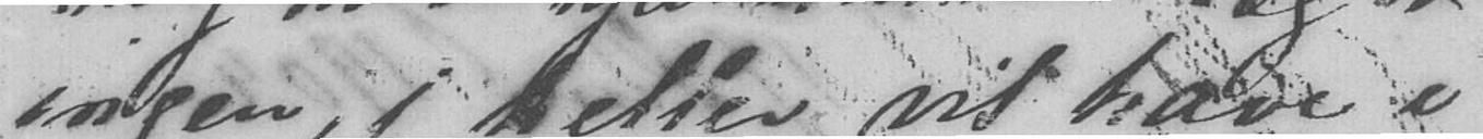ingen, jeg heller vil have i
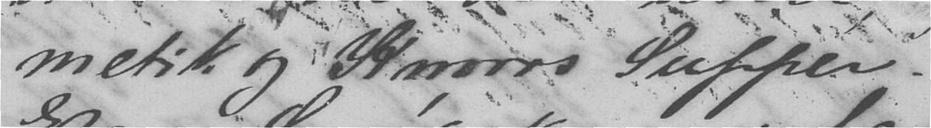metik og Knorrs Supper.
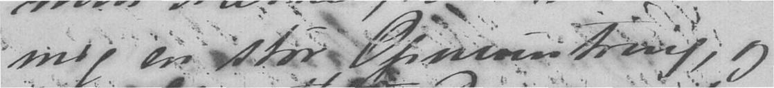mig en stor Opmuntring, og
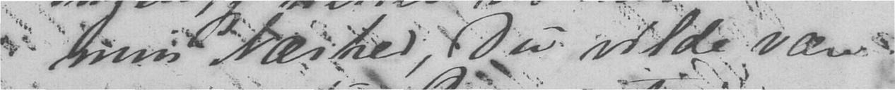min Nærhed, Du vilde være
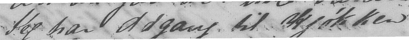Jeg har Adgang til Kjøkken
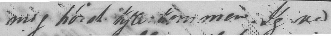mig høist kjærkommen. Jeg ved
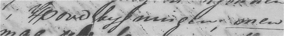i Hovedbygningen; men
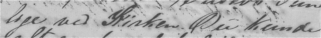lige ved Kirken. Du kunde
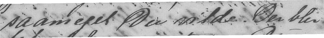saameget Du vilde. Der blir
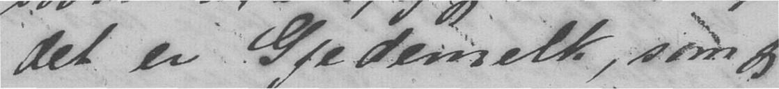det er Gjedemelk, som jeg
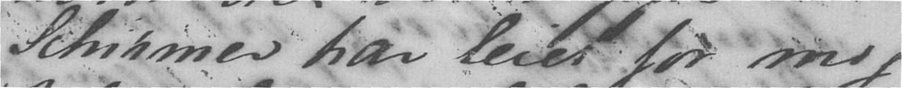Schirmer har leiet for mig
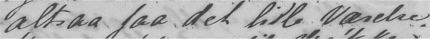altsaa faa det lille Værelse.
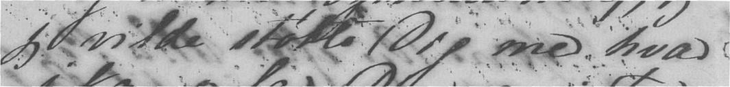jeg vilde støtte Dig med hvad
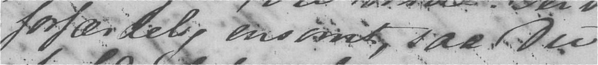forfærdelig ensomt, saa Du
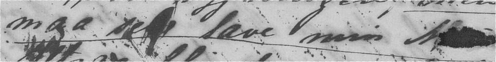maa selv lave min M
